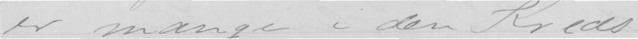er mange i den Kreds
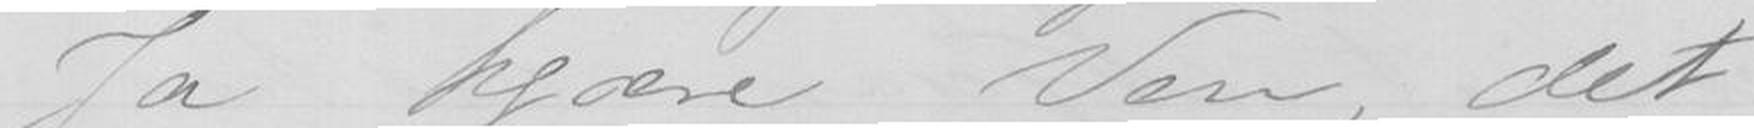Ja kjære Ven, det
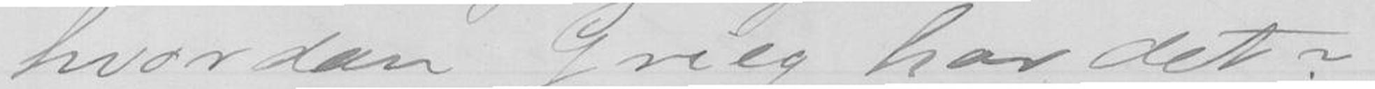hvordan Grieg har det?
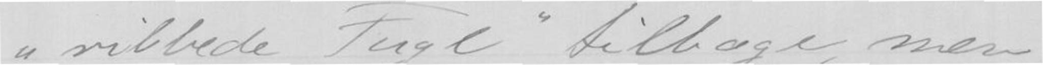ribbede Fugl tilbage, men
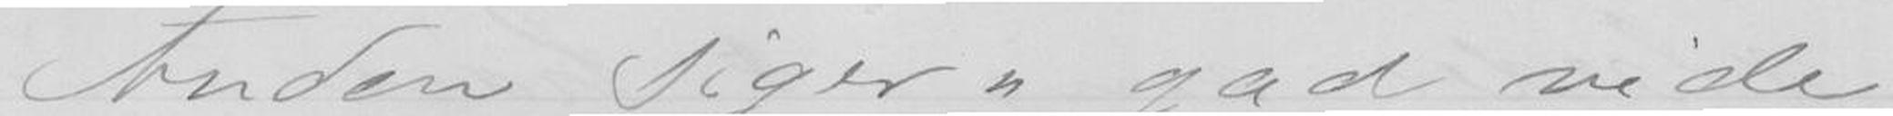Anden siger gad vide
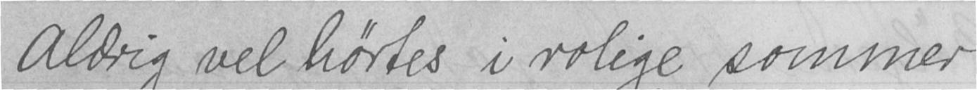Aldrig vel hørtes i rolige sommer
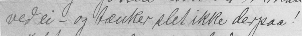ved ei - og tænker slet ikke derpaa!
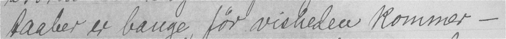taaber er bange, før visheden kommer -
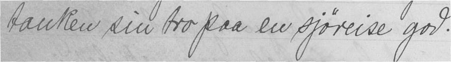tanken sin tro paa en sjøreise god.
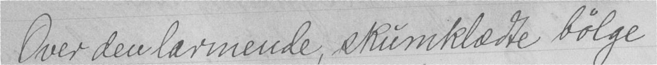Over den larmende, skumklædte bølge
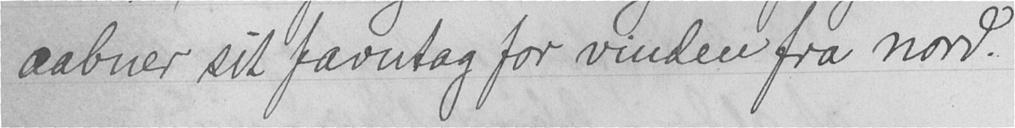aabner sit favntag for vinden fra nord.
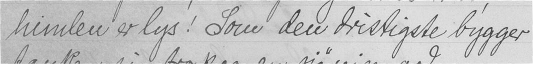himlen er lys! Som den dristigste bygger
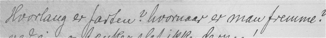Hvorlang er farten? hvornaar er man fremme?
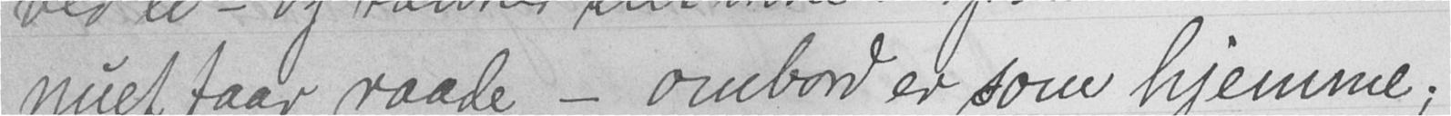nuet faar raade, - ombord er som hjemme;
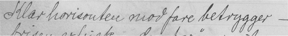Klar horisonten mod fare betrygger -
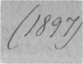(1897)
